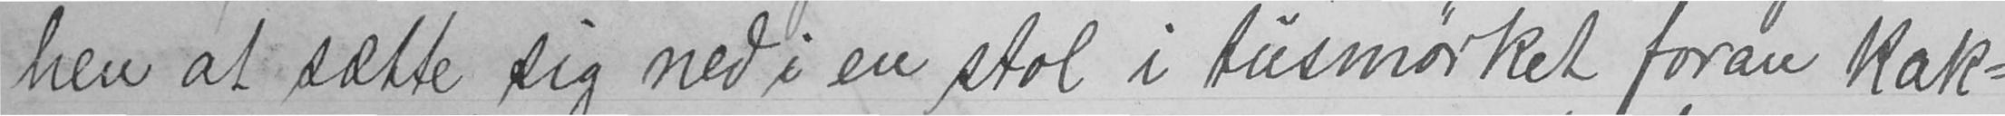hen at sætte sig ned i en stol i tusmørket foran kak-
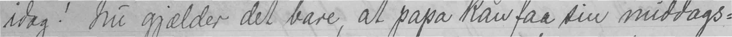idag! Nu gjælder det bare, at papa kan faa sin middags-
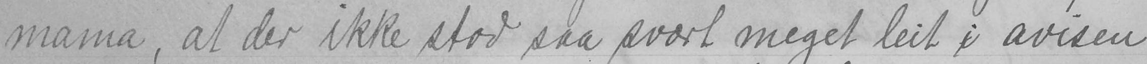mama, at der ikke stod saa svært meget leit i avisen
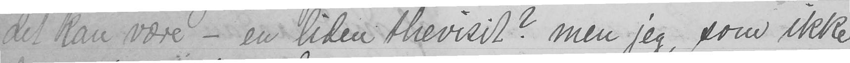det kan være - en liden thevisit? men jeg som ikke
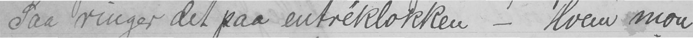Saa ringer det paa entréklokken - Hvem mon
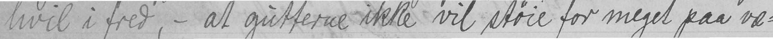hvil i fred, - at gutterne ikke vil støie for meget paa væ-
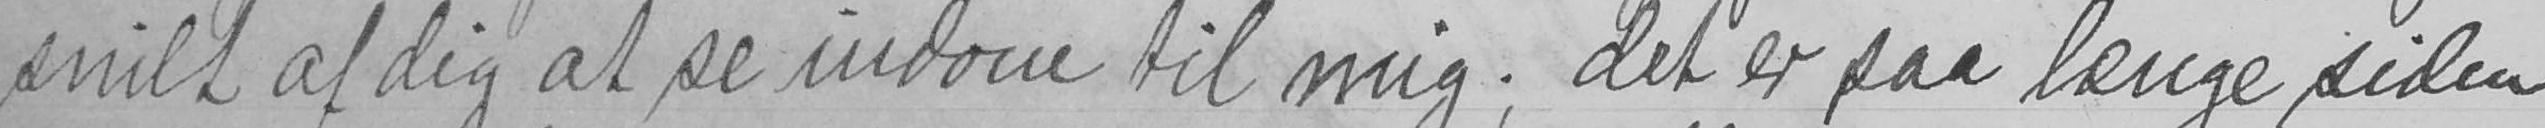snilt af dig at se indom til mig; det er saa lenge siden
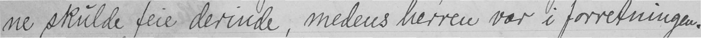ne skulde feie derinde, medens herren var i forretningen.
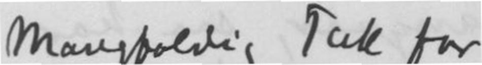Mangfoldig Tak for
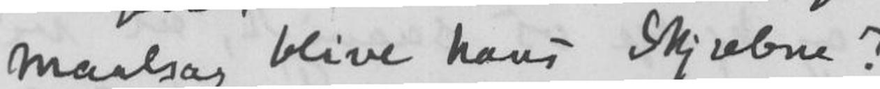Maalsag blive hans Skjæbne?
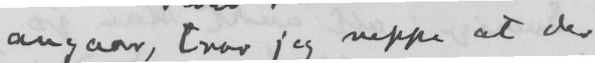angaar, tror jeg neppe at der
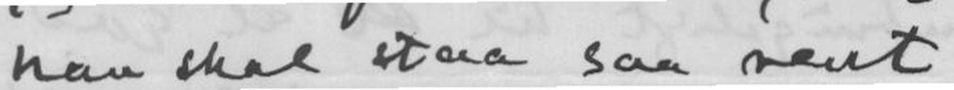han skal staa saa rent
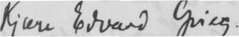Kjære Edvard Grieg.
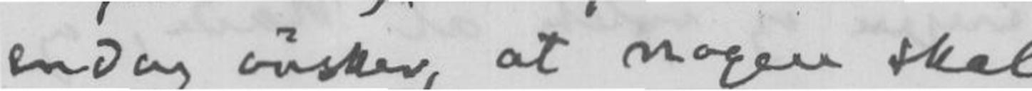endog ønsker, at nogen skal.
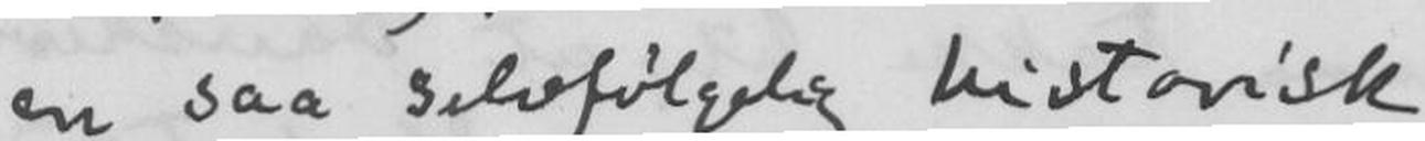en saa selvfølgelig historisk
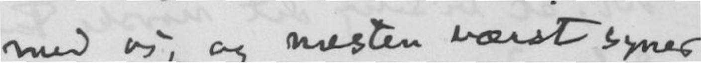med os, og nesten værst synes
